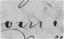om.
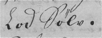Lod Sølv.
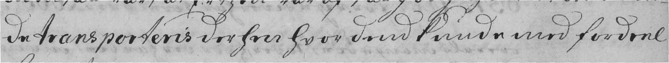de transporteris derhen hvor dend kunde med fordeel
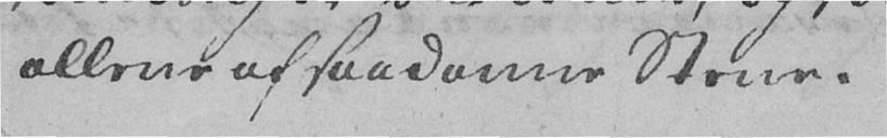allene af saadanne Stene.
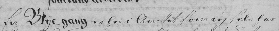En Blye-gang er her i Amtet som ieg selv har
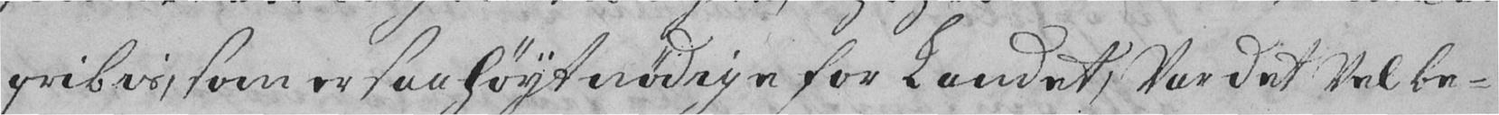gribis, som er saa høyt nødige for Landet, Var det Vel be-
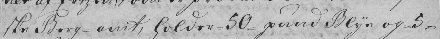ske Berg-amt, holder 50 pund Blye og 5
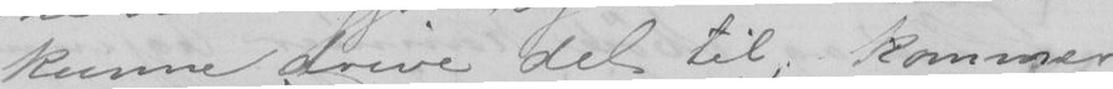kunne drive det til; kommer
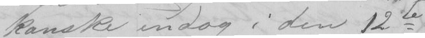Tkanske endog i den 12te.
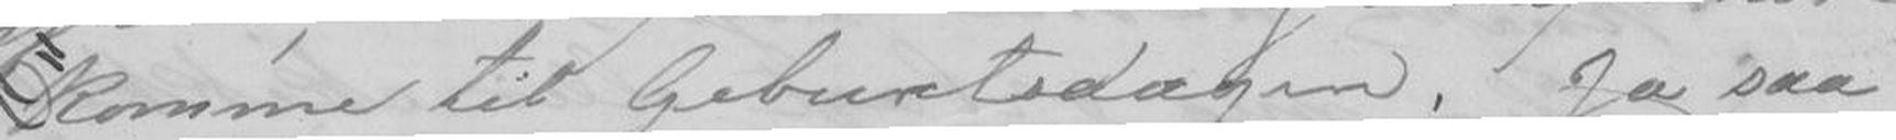komme til Geburtsdagen. Ja saa
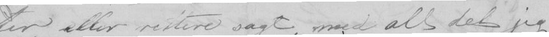ter, eller rettere sagt, med alt det jeg
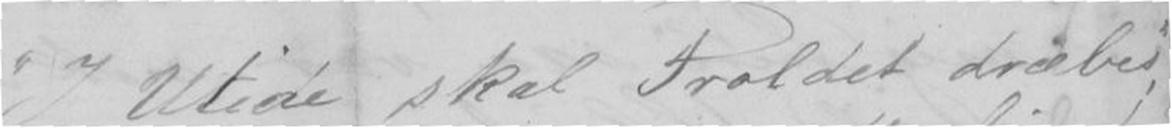I Utide skal Troldet dræbes,
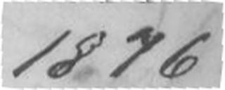1876
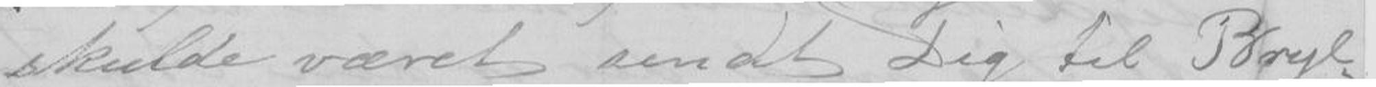skulde været sendt Dig til Bry-
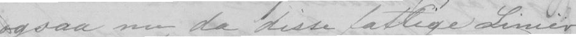ogsaa nu da disse fattige Linier,
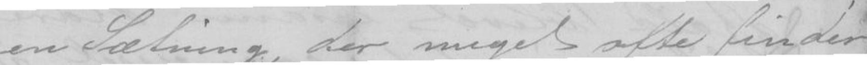en Sætning, der meget ofte finder
